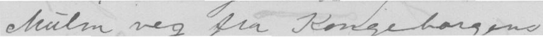Mulm veg fra Kongeborgens
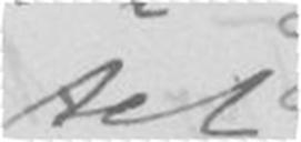tet
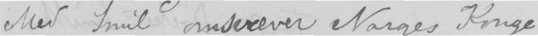Med Smil omsvæver Norges Konge
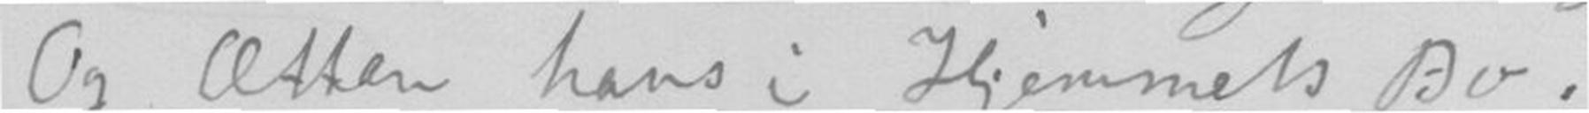Og Ætten hans i Hjemmets Bo.
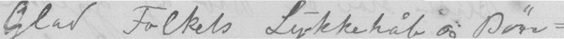Glad Folkets Lykkehåb og Bøn-
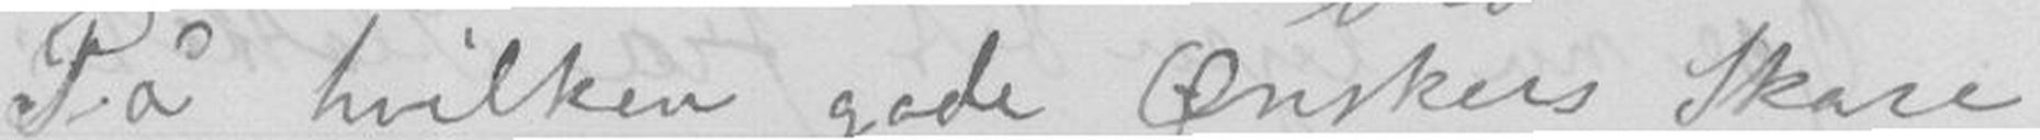På hvilken gode Ønskers Skare
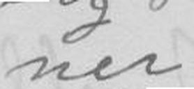ner
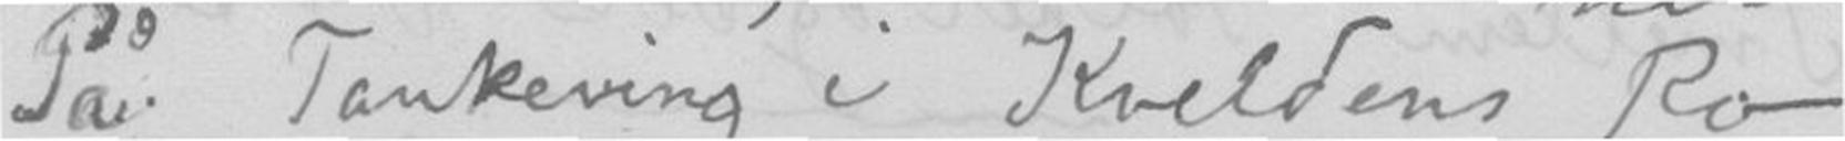På Tankeving' i Kveldens Ro
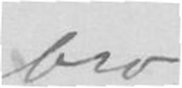bro
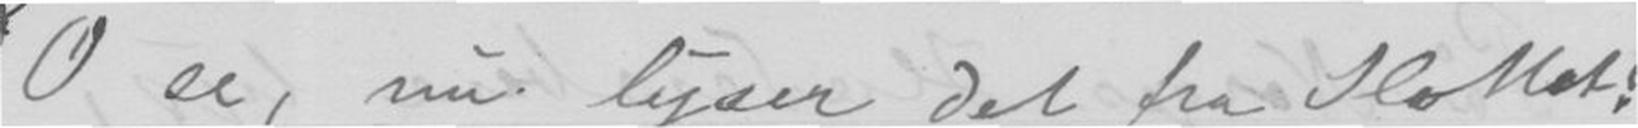O se, nu lyser det fra Slottet!
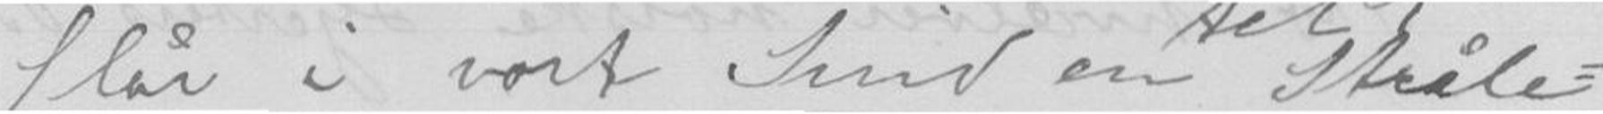Slår i vort Sind en Stråle-
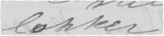lokker
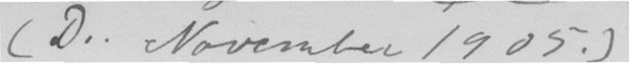(D. November 1905)
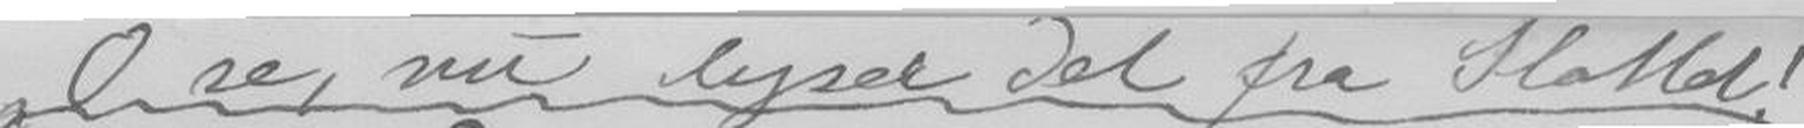O se, nu lyser det fra Slottet!
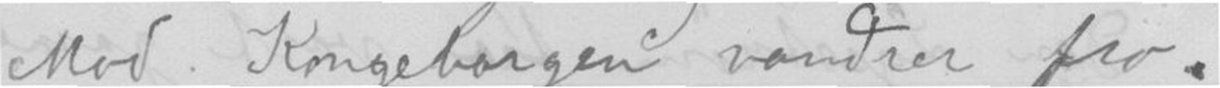Mod Kongeborgen vandrer fro.
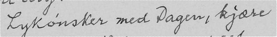Lykønsker med Dagen, kjære
